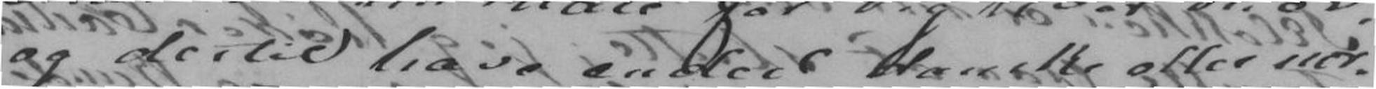og dertil have endeel danske eller nor-
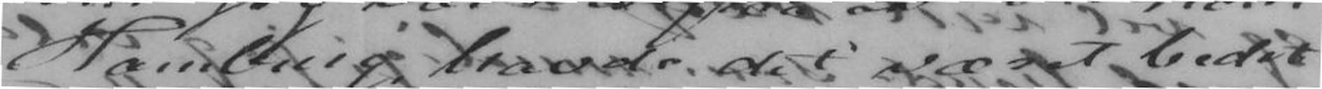til Hamburg, havde det været bedst
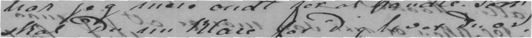skal Du nu klare for Dig hvor Du er,
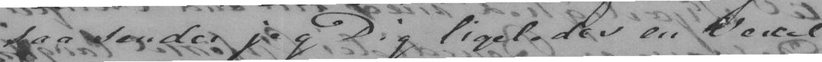saa sender jeg Dig ligeledes en Vexel
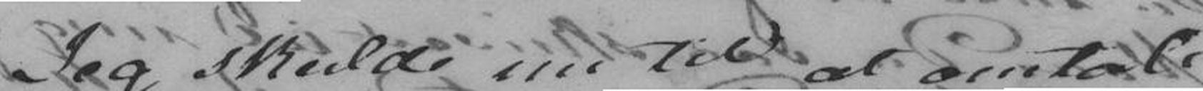Jeg skulde nu til at omtale
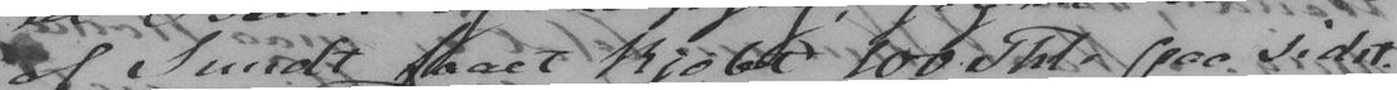af Sundt faaet kjøbt 100Thl paa sidst
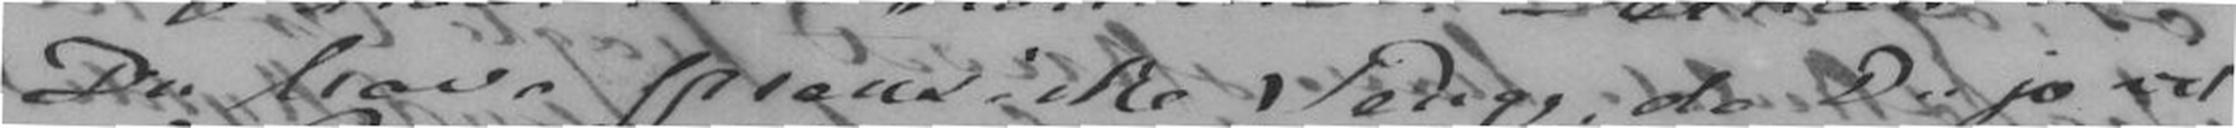Du have Preusiske Penge, da Du jo vil
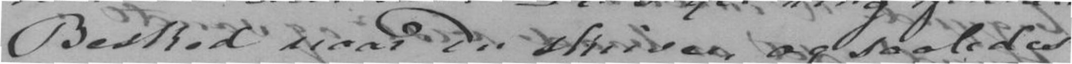Besked naar Du skriver og saaledes
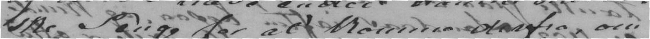ske Penge for at komme derfra, om
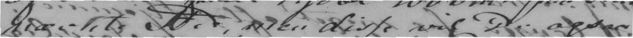nævnte Tur, men disse vil Du ogsaa
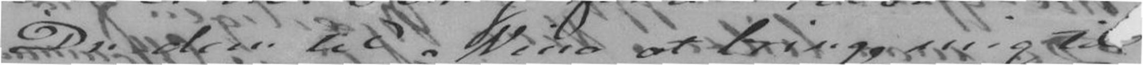Du dem til Nina at bringe mig til-
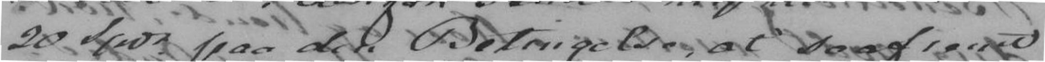20Spdo paa den Betingelse at saafremt
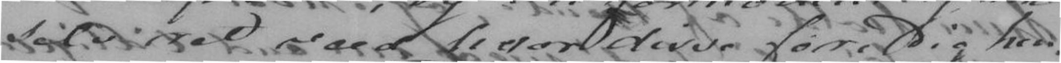selv ret veed hvor disse føre Dig hen,
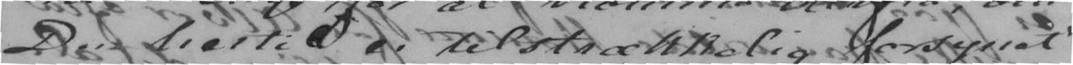Du hertil er tilstrækklig forsynet
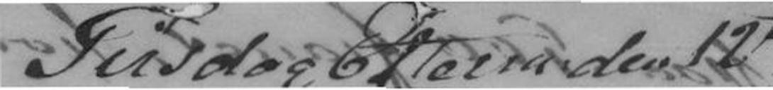Tirsdag Efterm. den 12te.
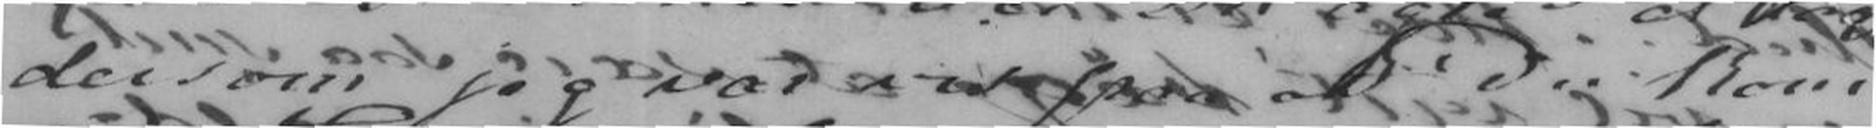dersom jeg var vis paa at Du kom
